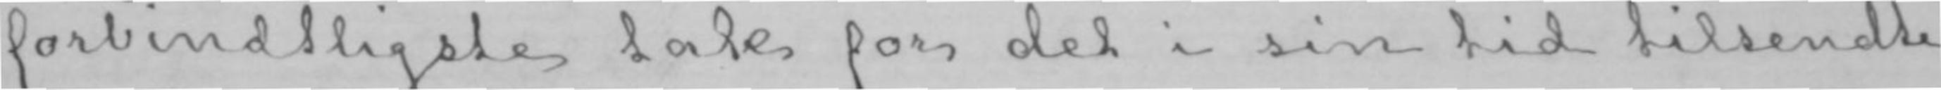forbindtligste tak for det i sin tid tilsendte
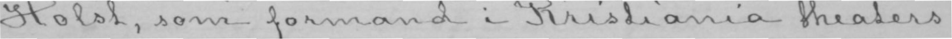Holst, som formand i Kristiania theaters
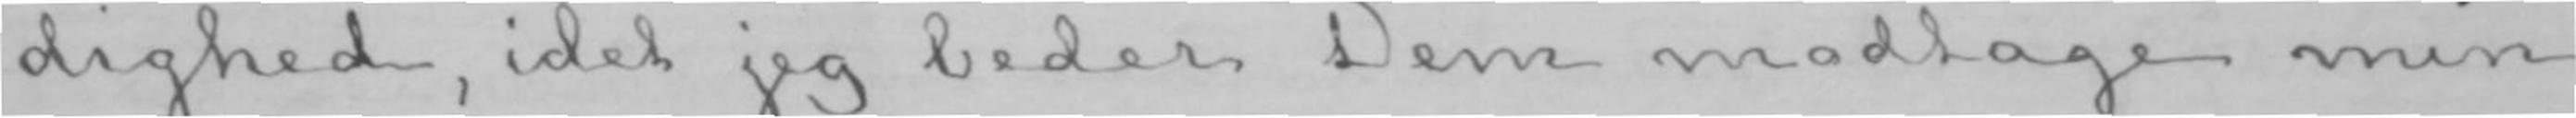dighed, idet jeg beder Dem modtage min
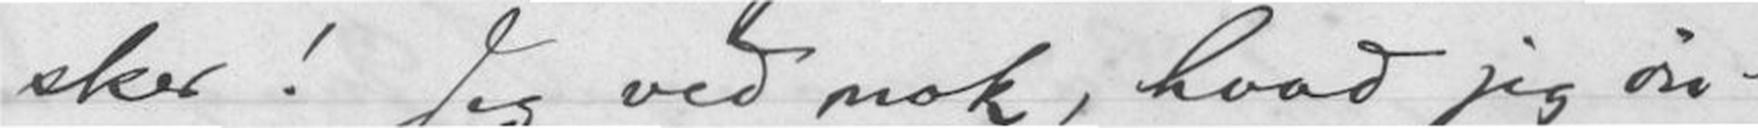sker! Jeg ved nok, hvad jeg øn-
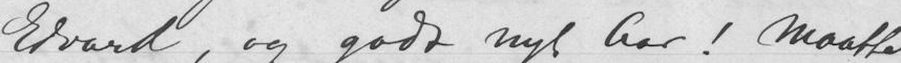Edvard, og godt nyt Aar! Maatte
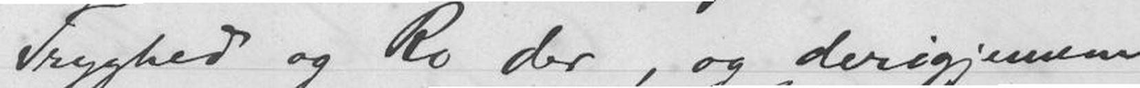Tryghed og Ro der, og derigjennem
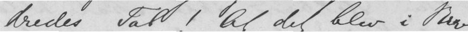dredes Tal! At det blev i Brev
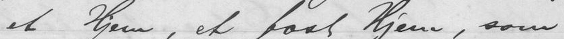et Hjem, et fast Hjem, som
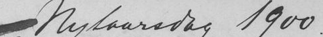Nytaarsdag 1900
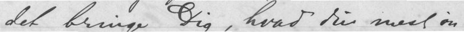det bringe Dig, hvad du mest øn-
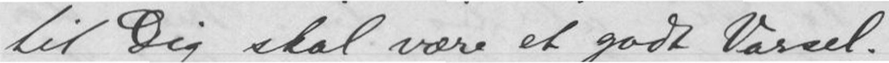til Dig skal være et godt Varsel.
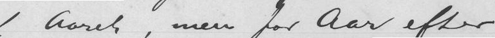af Aaret, men for Aar efter
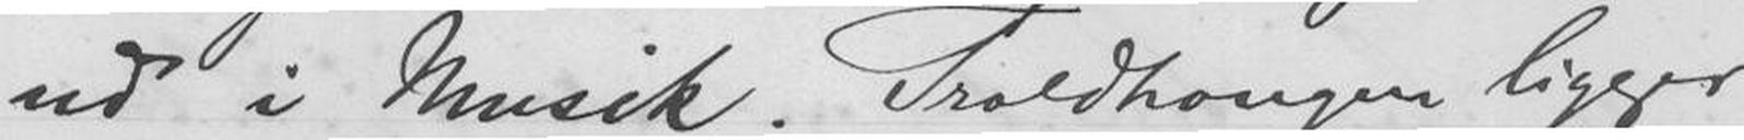ud i Musik. Troldhaugen ligger
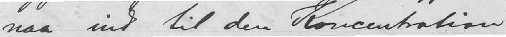naa ind til den Koncentration
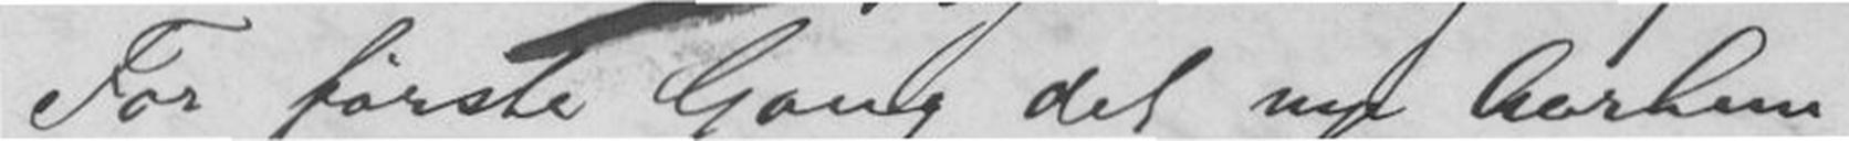For første Gang det nye Aarhun-
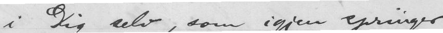i Dig selv, som igjen springer
